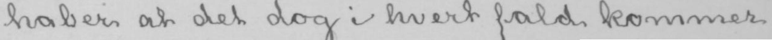håber at det dog i hvert fald kommer
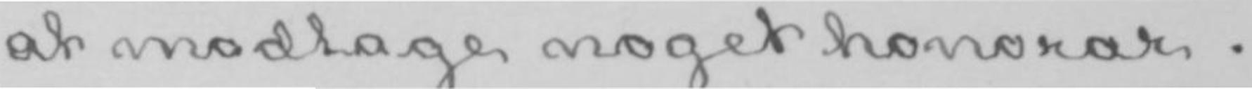at modtage noget honorar.
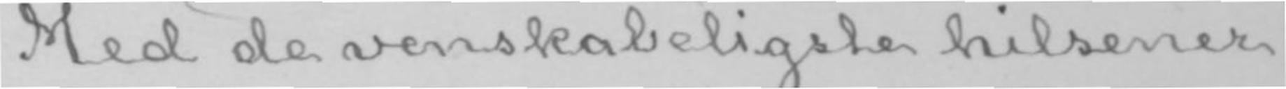Med de venskabeligste hilsener
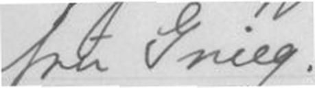fru Grieg.
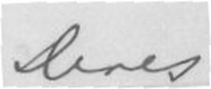Deres
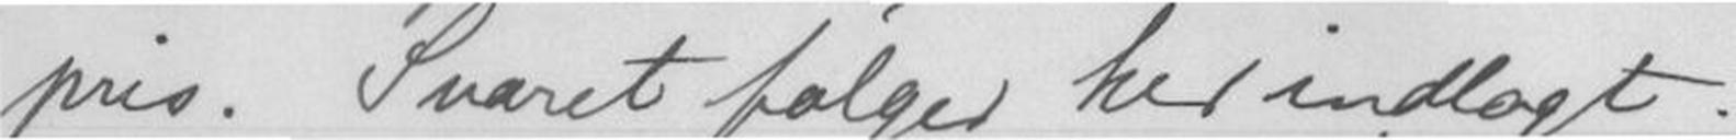pris. Svaret følger her indlagt.
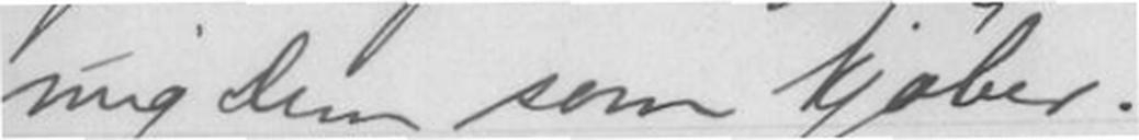mig Dem som kjøber.
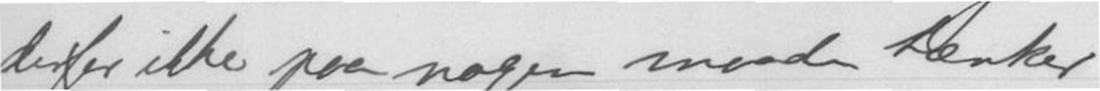derfor ikke paa nogen maade tænker
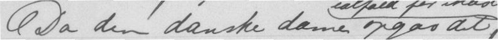Da den danske dame opgav det ialfald for ihøst
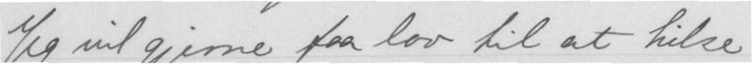Jeg vil gjerne faa lov til at hilse
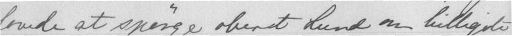lovede at spørge oberst Lund om billigste
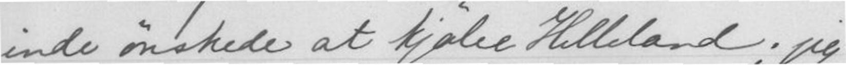inde ønskede at kjøbe Helleland; jeg
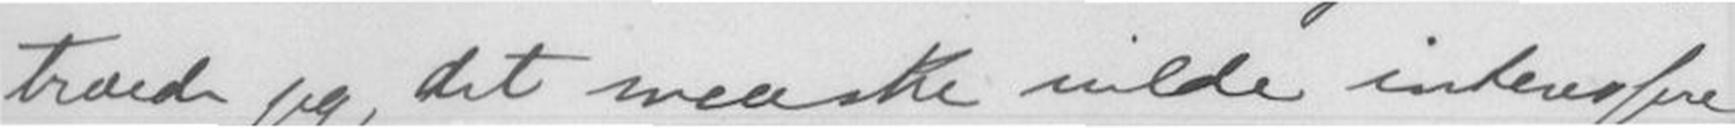troede jeg, det maaske vilde interessere
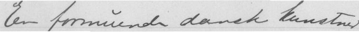En formuende dansk kunstner-
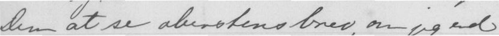Dem at se oberstens brev, om jeg end
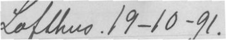Lofthus. 19-10-91.
